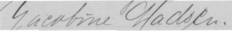Jacobine Madsen
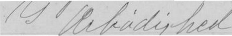I Ærbødighed
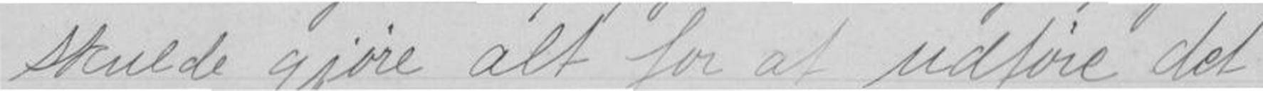skulde gjöre alt for at udföre det
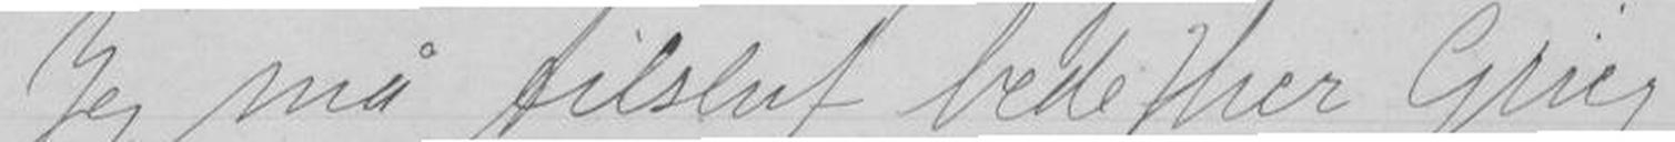Jeg må tilslut bede Herr Grieg
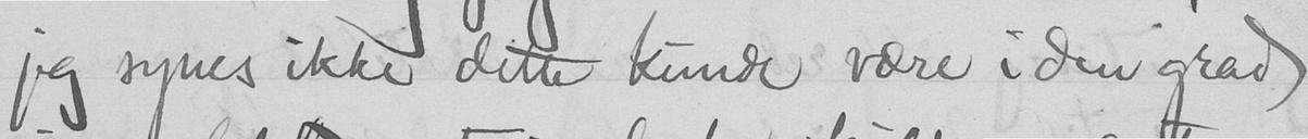jeg synes ikke dette kunde være i den grad
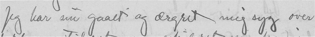Jeg har nu gaaet og ærgret mig syg over
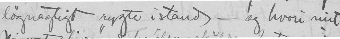løgnagtigt rygte i stand - og hvori mit
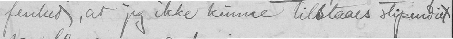fenhed, at jeg ikke kunne tilstaaes stipendiet
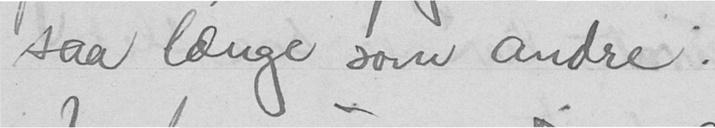saa længe som andre:
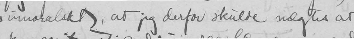umokalskt, at jeg derfor skulde nægtes at
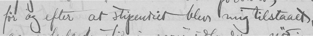for og efter at stipendiet blev tig tilstaaet,
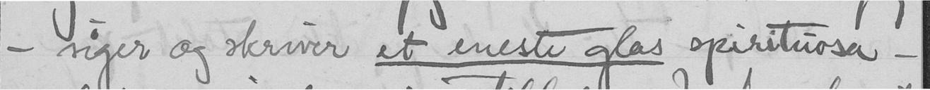siger og skriver et eneste glas spirituosa -
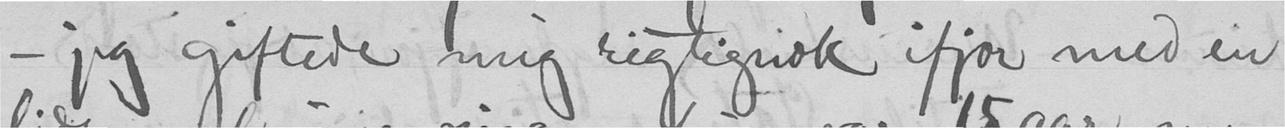- jeg giftede mig rigtignok ifjor med en
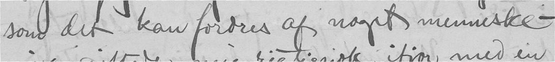som det kan fordres af noget menneske-
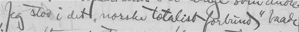Jeg stod i det "norske totalist forbund" baade
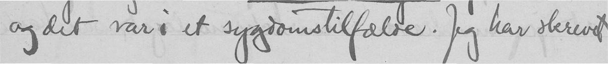og det var i et sygdomstilfælde. Jeg har skrevet
